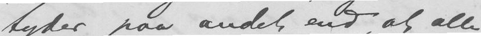tyder paa andet end, at alle
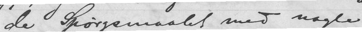de Spørgsmaalet med nogle
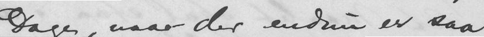Dage, naar der endnu er saa
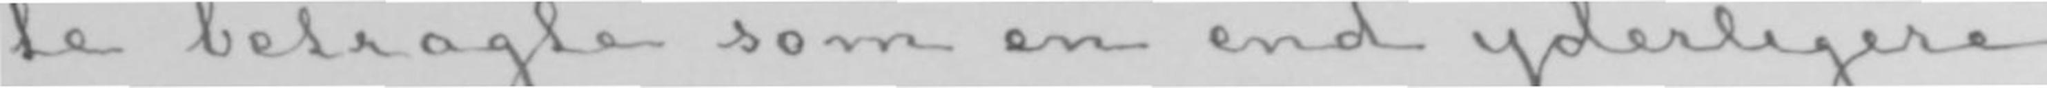te betragte som en end yderligere
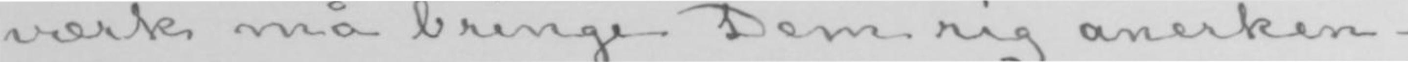værk må bringe Dem rig anerken-
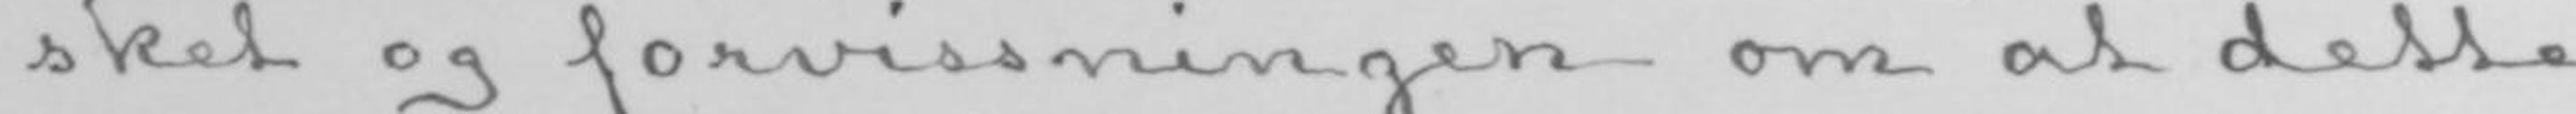sket og forvissningen om at dette
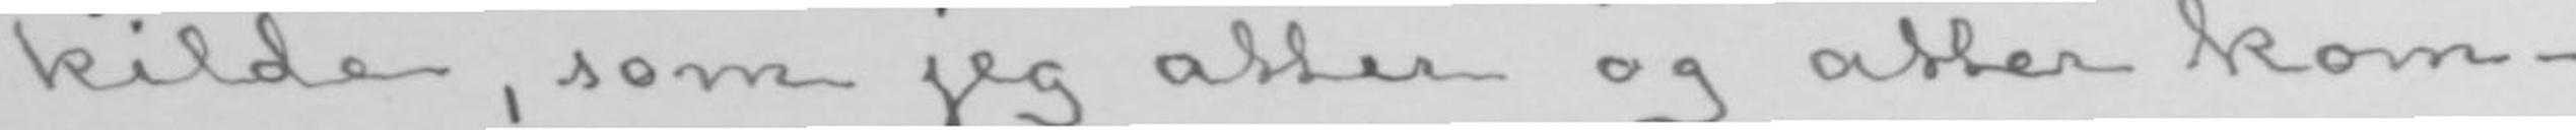kilde, som jeg atter og atter kom-
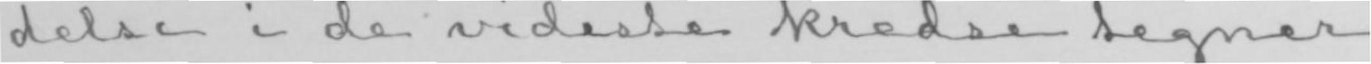delse i de videste kredse tegner
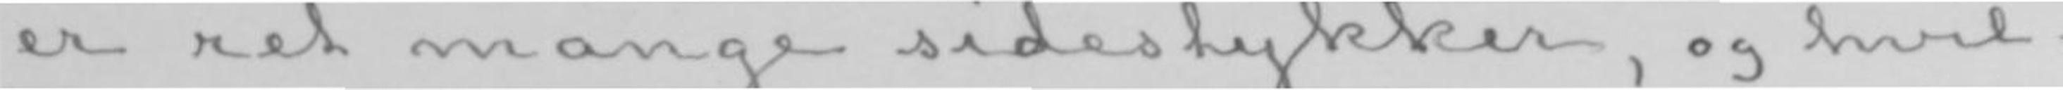er ret mange sidestykker, og hvil-
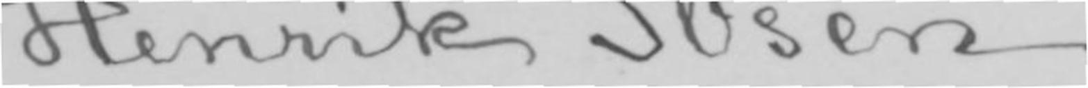Henrik Ibsen.
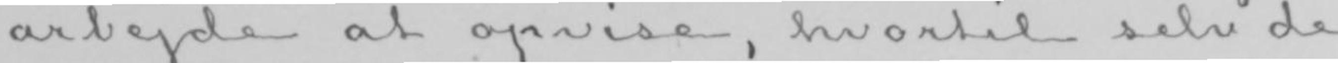arbejde at opvise, hvortil selv de
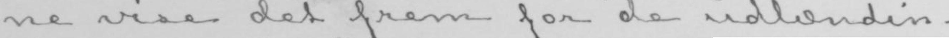ne vise det frem for de udlændin-
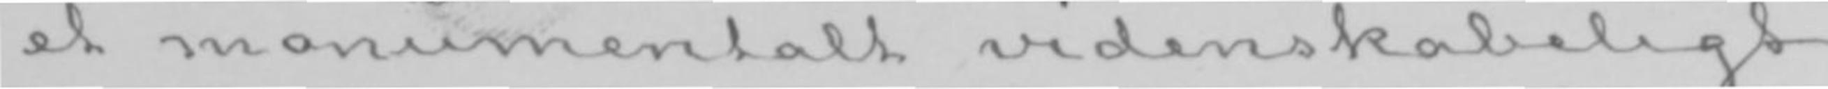et monumentalt videnskabeligt
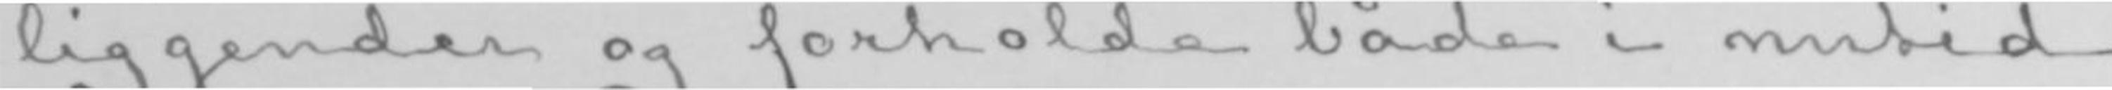liggender og forholde både i nutid
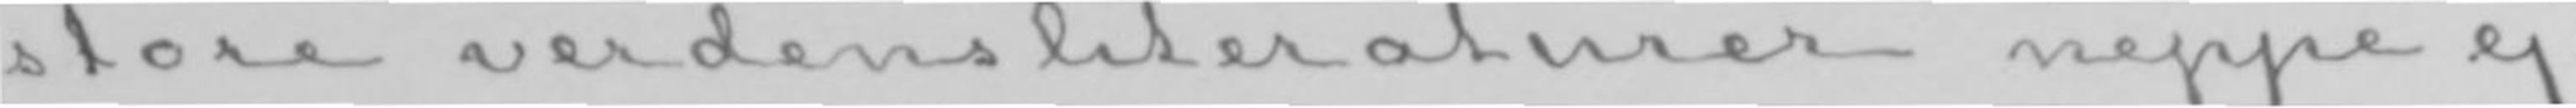store verdensliteraturer neppe ej-
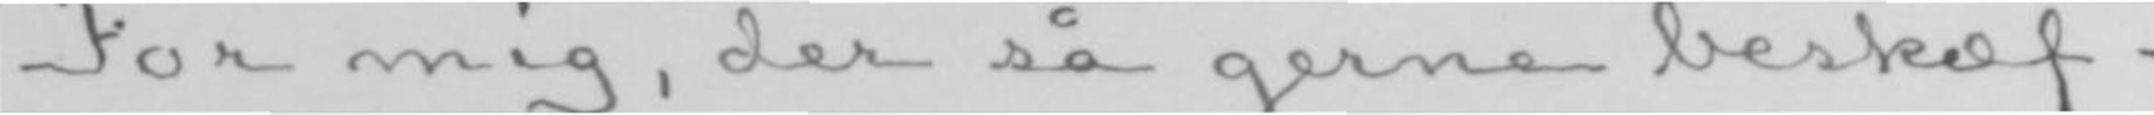For mig, der så gerne beskæf-
